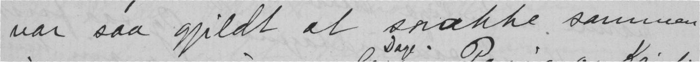var saa gjildt at snakke sammen
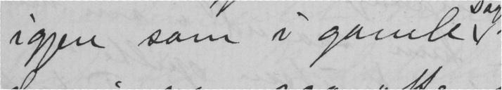igjen som i gamle .
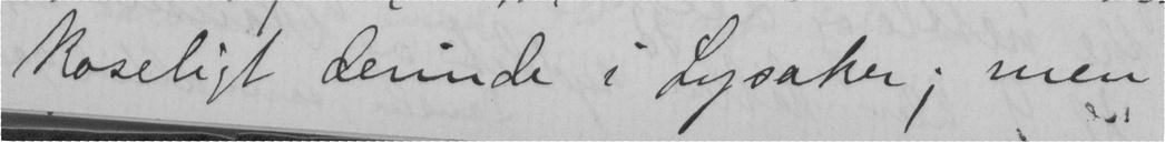koseligt derinde i Lysaker; men
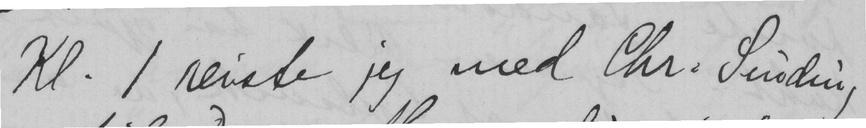Kl. 1 reiste jeg med Chr. Sinding
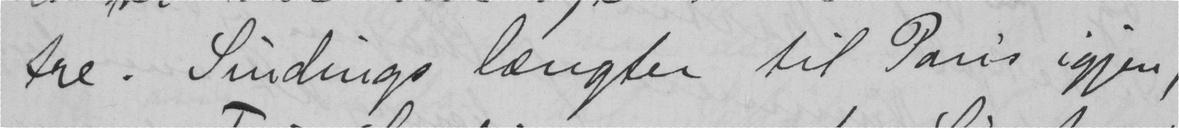tre. Sindings længter til Paris igjen,
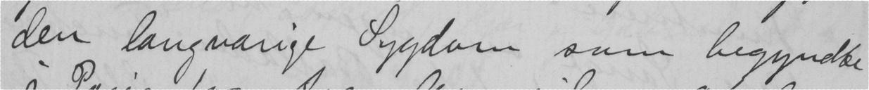den langvarige sygdom som begyndte
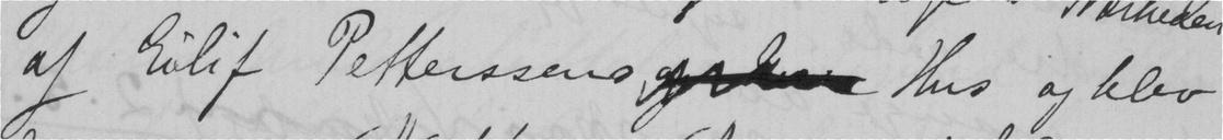af Eilif Petterssens  Hus og blev
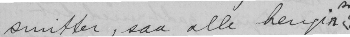smitter, saa alle hengir
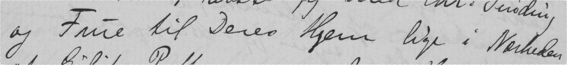og Frue til Deres Hjem lige i Nærheden
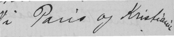i Paris og Kristiania
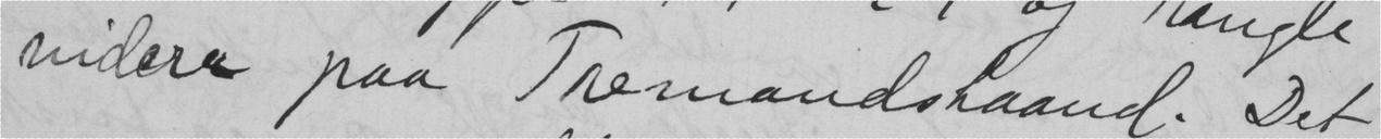videre paa Tremandshaand. Det
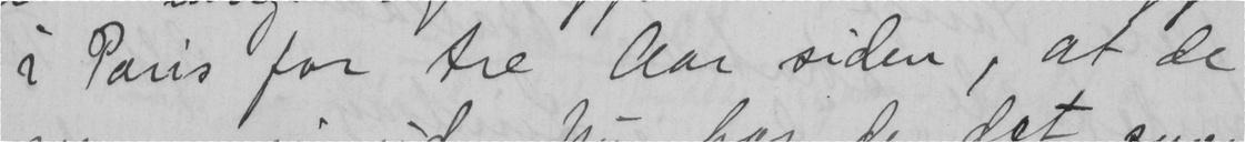i Paris for tre Aar siden, at de
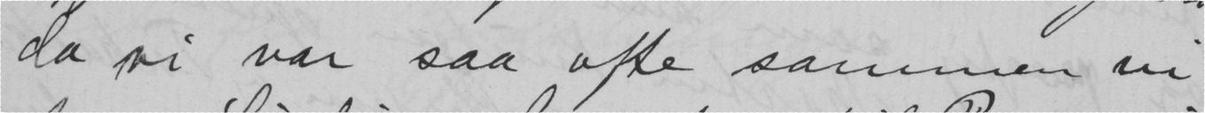da vi var saa ofte sammen vi
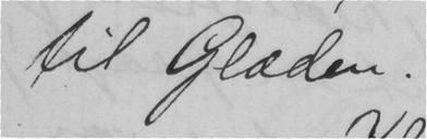til Glæden.
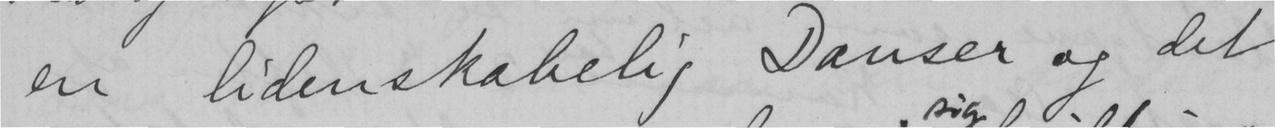en lidenskabelig Danser og det
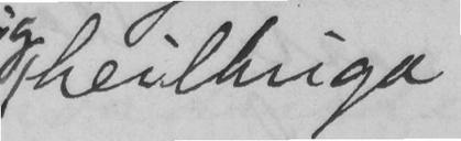heilhuga
